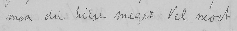maa du hilse meget Vel mødt
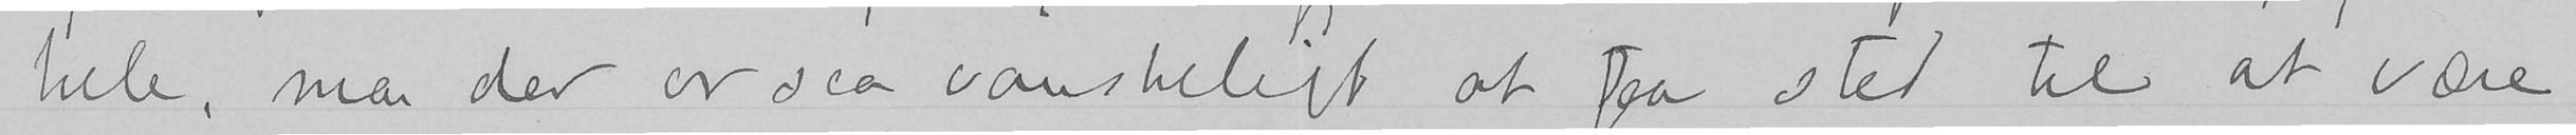hele, men der er saa vanskeligt at faa sted til at være,
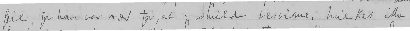feil, for han var ræd for, at jeg skulde besvime, hvilket ikke
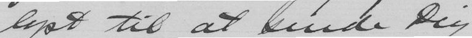lyst til at sende Dig
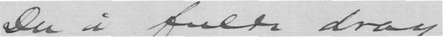Du i fulde drag
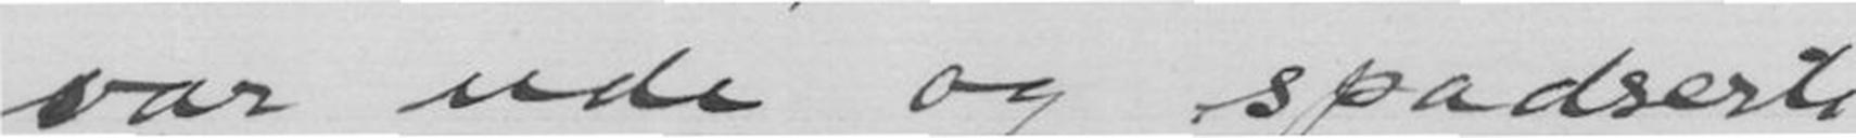var ude og spadserte
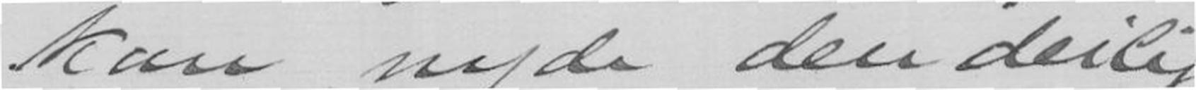kan nyde den deilig
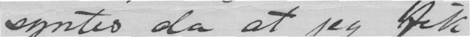syntes da at jeg fik
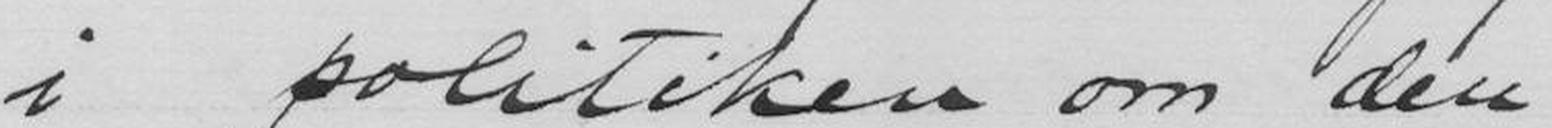i politiken om den
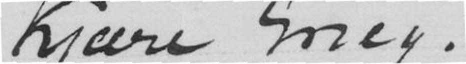Kjære Grieg!
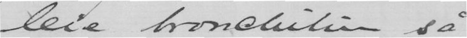leie bronchitten så
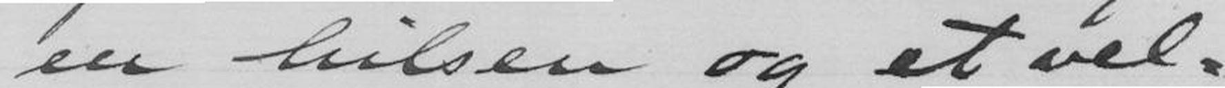en hilsen og et vel-
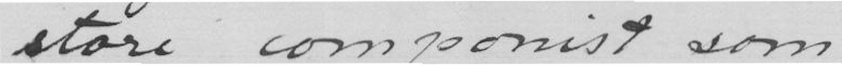store componist som
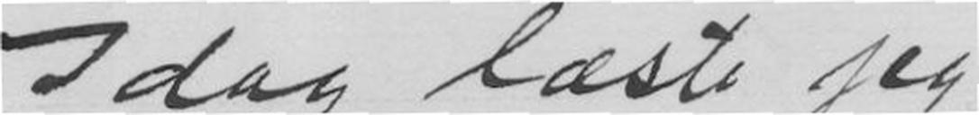I dag læste jeg
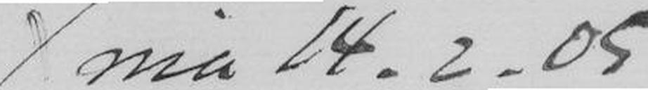Xnia 14.2.05
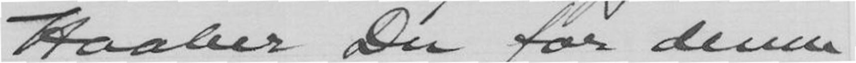Haaber Du for denne
